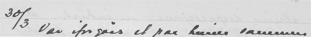30/3 Var iforgårs et par timer sammen
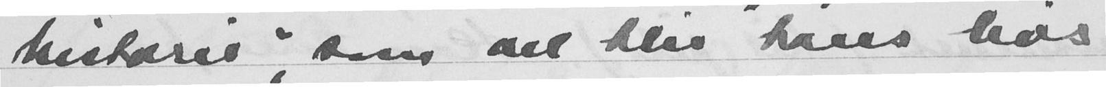historie som vel blir hans livs
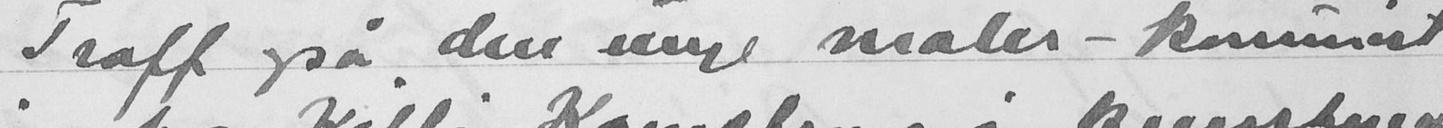Traff også den unge maler-komunist
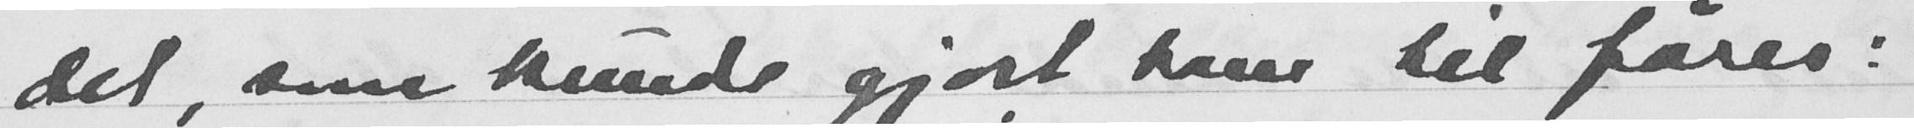det, som kunde gjort han til fører:
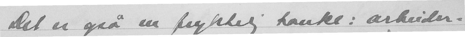Det er også en fryktelig tanke; arbeider-
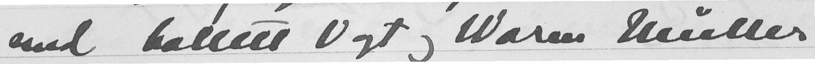med Collett Vogt og Waren Muller
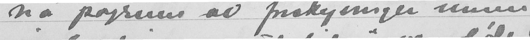nå pågrunn av forskyvninger innen
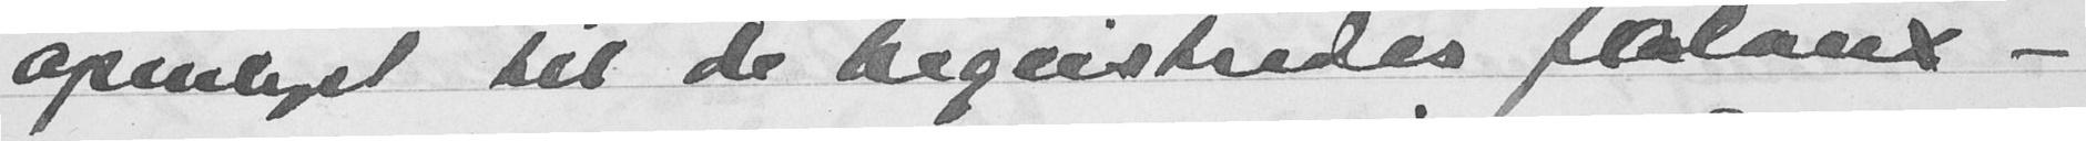åpenlyst til de begeistredes falaux -
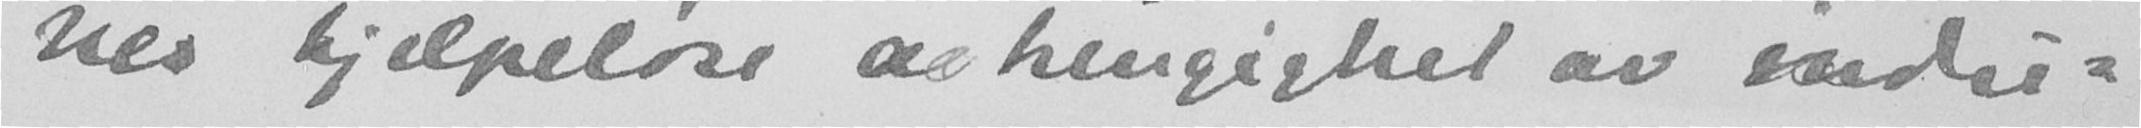nes hjelpeløse avhengighet av indu-
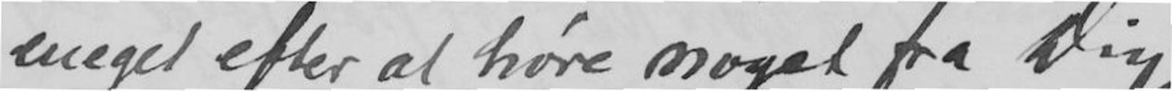meget efter at høre noget fra Dig;
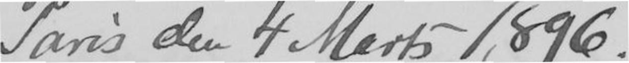Paris den 4 Marts 1896.
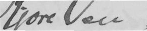Kjære Ven.
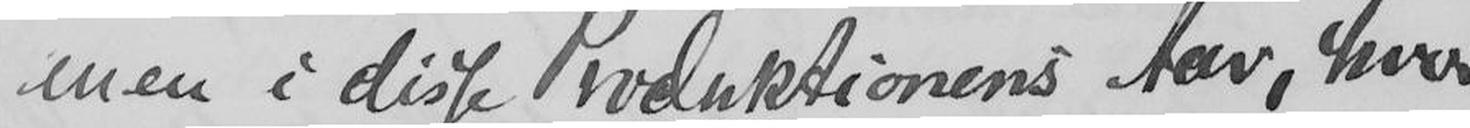men i disse Produktionens Aar, hvor
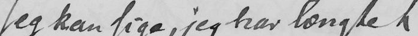Jeg kan sige, jeg har længtet
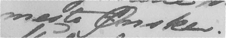meste Ønsker.
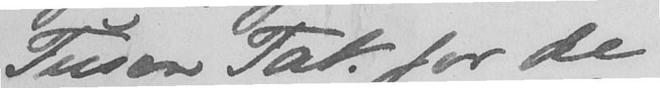Tusen Tak for de
